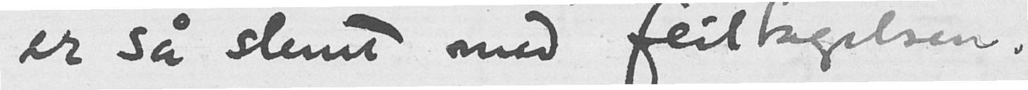er så slemt med feiltagelsen.
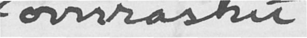overrasket
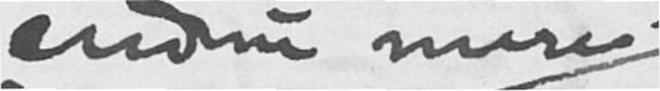endnu mere
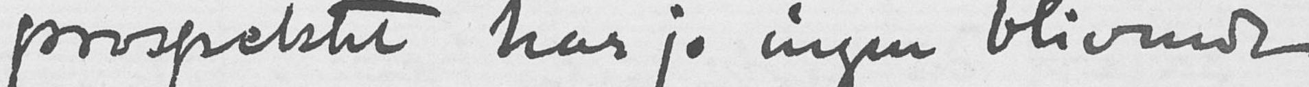prospektet har jo ingen blivende
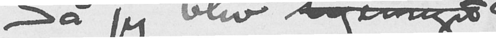Så jeg blev
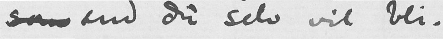som end du selv vil bli.
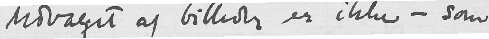Udvalget af billeder er ikke - som
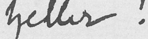heller!
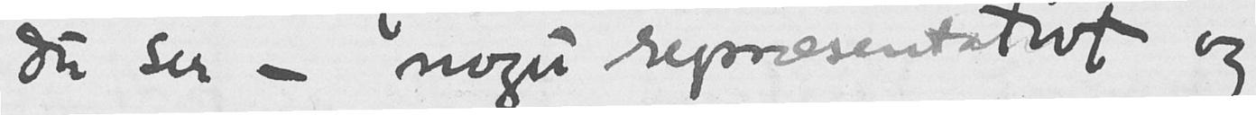du ser - noget repræsentativt og
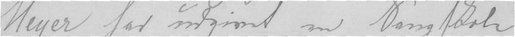Meyer har udgivet en Sangskole,
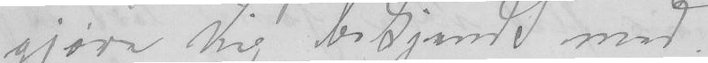gjøre Dig bekjendt med.
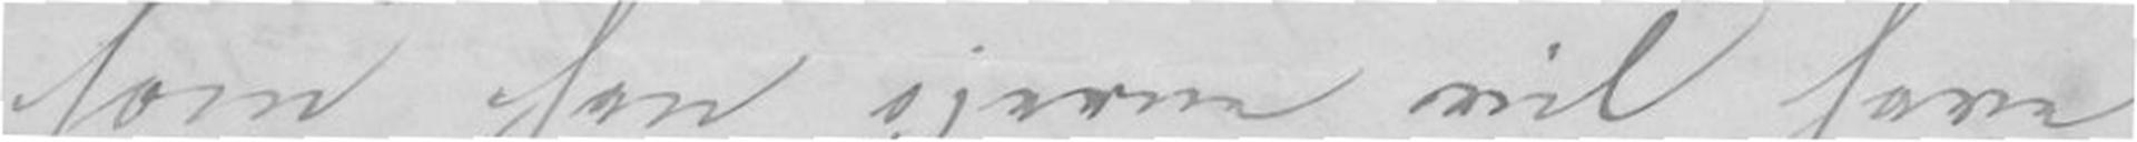som han gjerne vil have
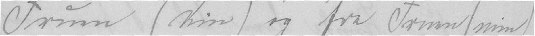Fruen (Din) og fra Fruen (min)
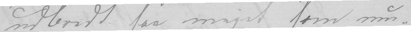udbredt saa meget som mu-
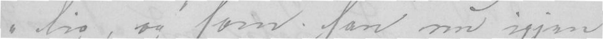lig, og som han nu igjen-
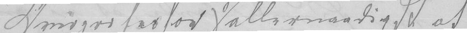Overprofessor allernaadigst at
